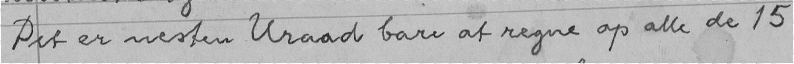Det er nesten Uraad bare at regne op alle de 15
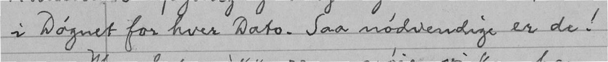i Døgnet for hver Dato. Saa nødvendige er de!
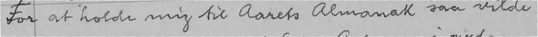For at holde mig til Aarets Almanak saa vilde
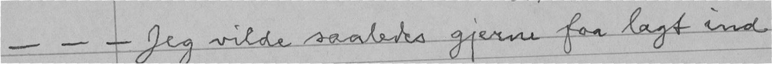- - - Jeg vilde saaledes gjerne faa lagt ind
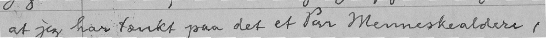at jeg har tænkt paa det et Par Menneskealdere,
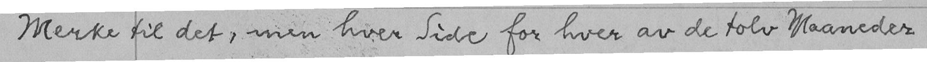Merke til det, men hver Side for hver av de tolv Maaneder
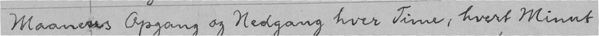Maanens Opgang og Nedgang hver Time, hvert Minut
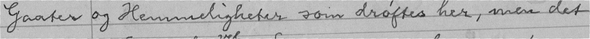Gaater og Hemmeligheter som drøftes her, men det
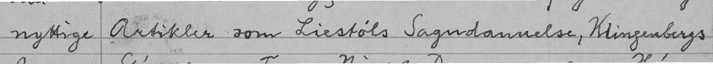nyttige Artikler som Liestøls Sagndannelse, Klingenbergs
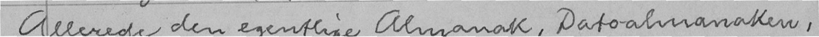Allerede den egentlige Almanak, Datoalmanaken,
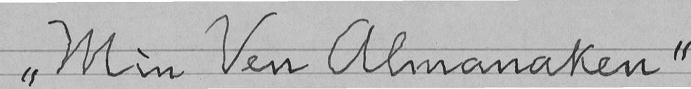"Min Ven Almanaken"
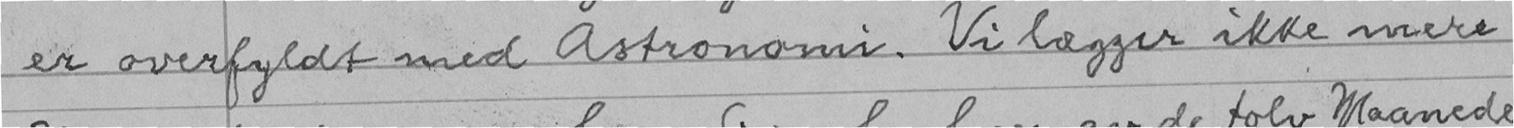er overfyldt med Astronomi. Vi lægger ikke mere
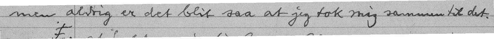men aldrig er det blit saa at jeg tok mig sammen til det.
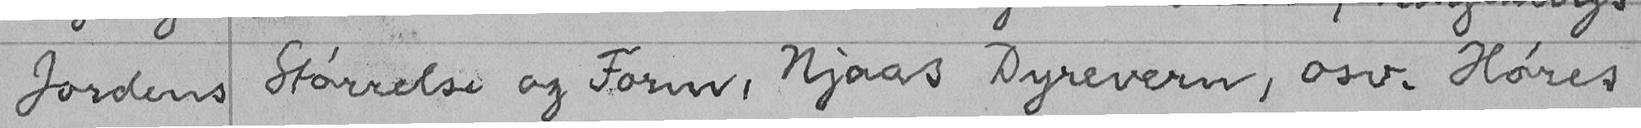Jordens Størrelse og Form, Njaas Dyrevern, osv. Høres
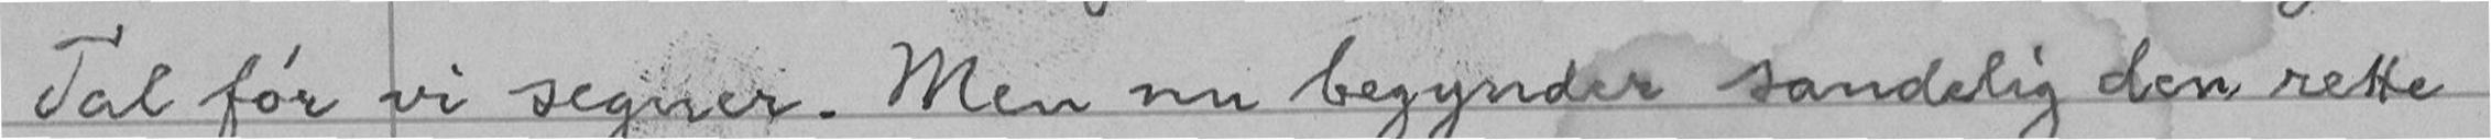Tal før vi segner. Men nu begynder sandelig den rette
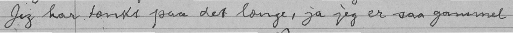Jeg har tænkt paa det længe, ja jeg er saa gammel
